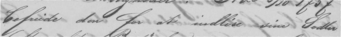befreide den for at indløse sine Sedler
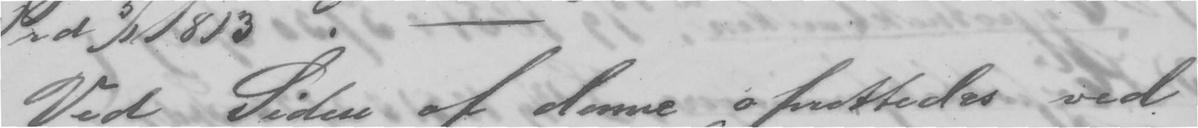Ved Siden af denne oprettedes ved
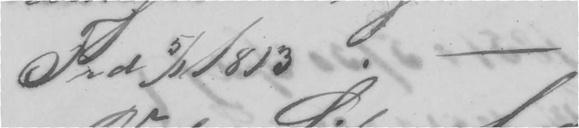Frd 5/1 1813. -
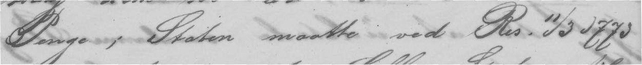Penge; Staten maatte ved Res. 11/3 1773
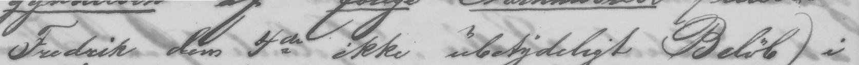Fredrik den 4de ikke ubetydeligt Beløb)
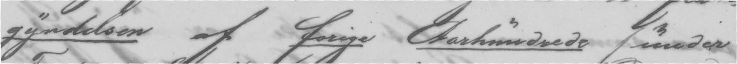gyndelsen af forige Aarhundrede (under
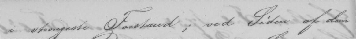i strengeste Forstand; ved Siden af dem
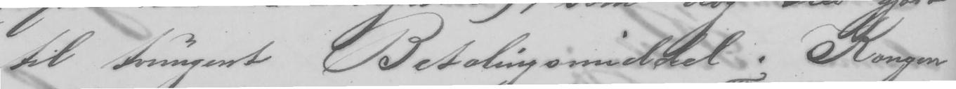til tvungent Betalingsmiddel. Kongen
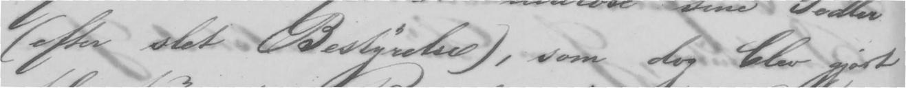(efter slet Bestyrelse), som dog blev gjort
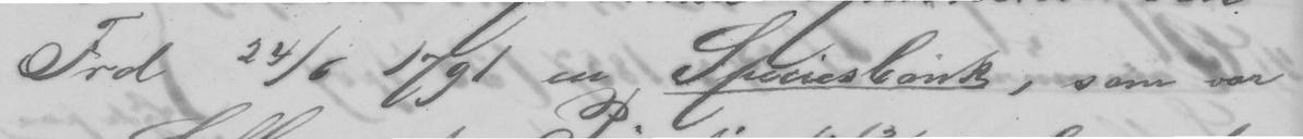Frd 24/6 1791 en Speciesbank, som var
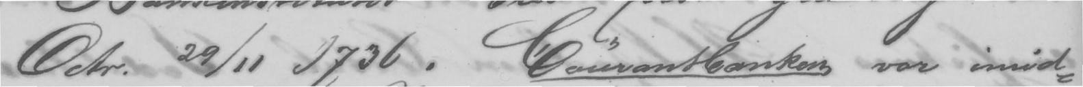Octr. 29/11 1736.  Courantbanken var imid-
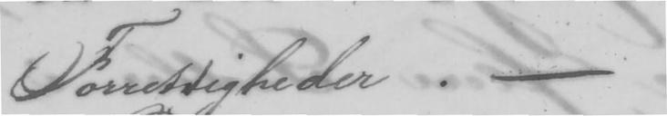Førrettigheder. -
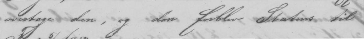overtage den, o den forblev Statens til
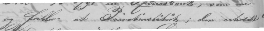og forblev et Privatinstitut; den erholdt
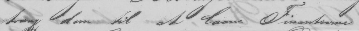tvang dem til at laane Finantserne
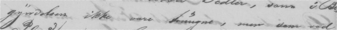gyndelsen ikke vare tvungne, men som ved
